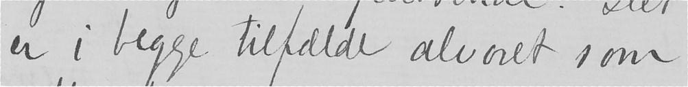er i begge tilfælde alvoret som
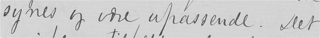synes og være upassende. Det
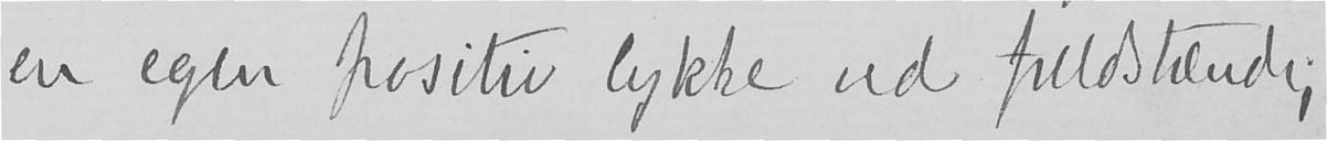en egen positiv lykke ved fuldstændig
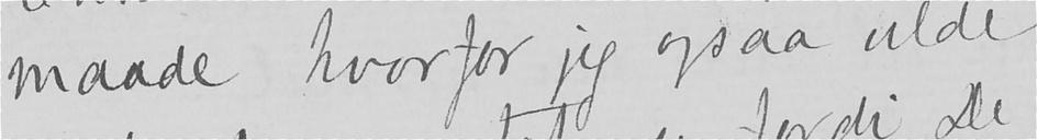maade hvorfor jeg ogsaa vilde
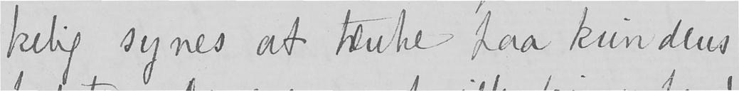kelig synes at tænke paa kvindens
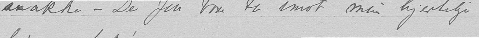snakke – De faar bare ta imot min hjertelige
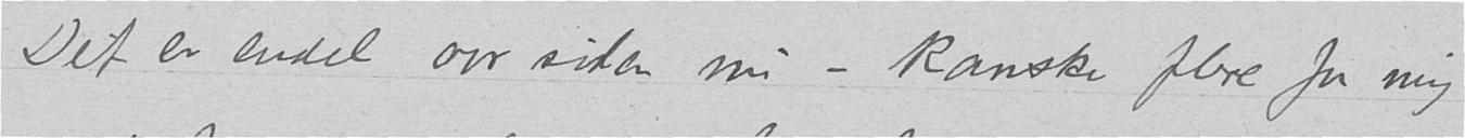Det er endel aar siden nu – kanske flere for mig
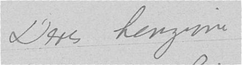Deres hengivne
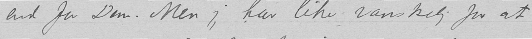end for Dem. Men jeg har like vanskelig for at
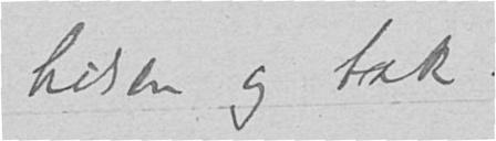hilsen og tak!
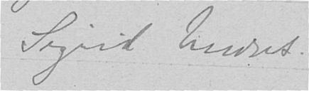Sigrid Undset.
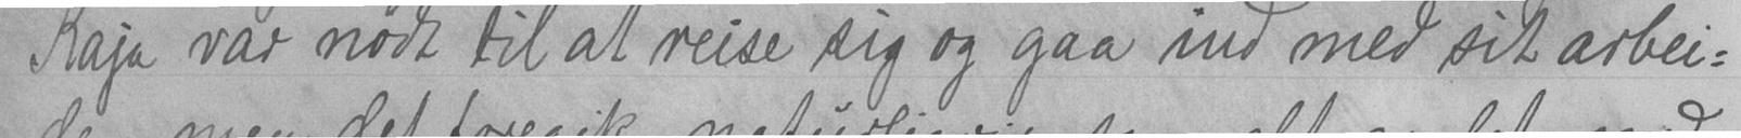Kaja var nødt til at reise sig og gaa ind med sit arbei-
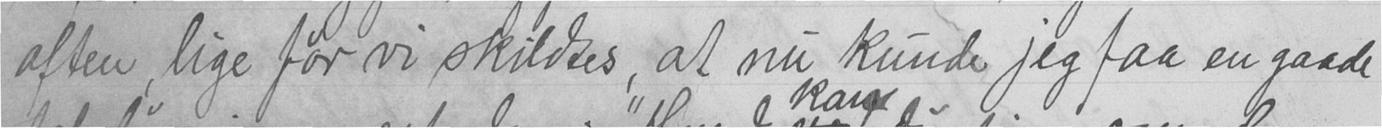aften, lige før vi skildtes, at nu kunde jeg faa en gaade
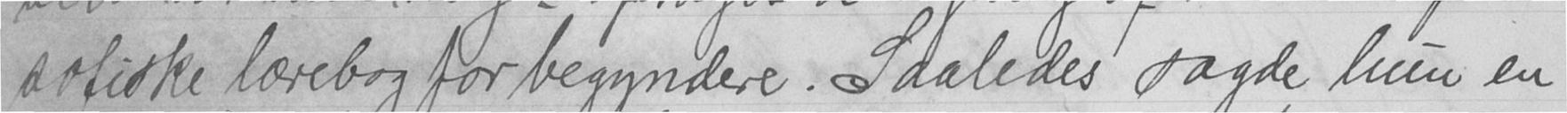sofiske lærebog for begyndere. Saaledes sagde hun en
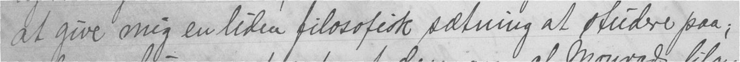at give mig en liden filosofisk sætning at studere paa;
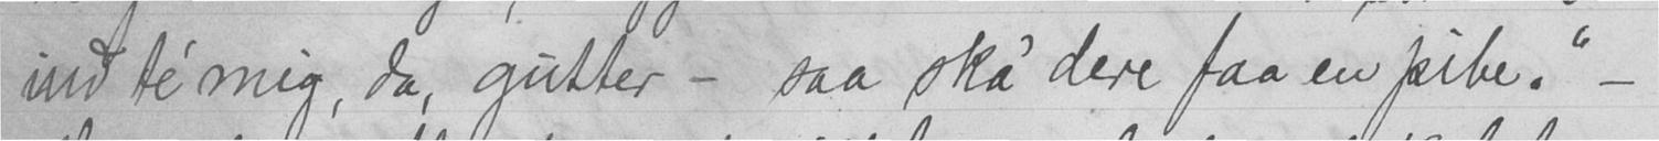ind te' mig, da, gutter - saa ska' dere faa en pibe." -
